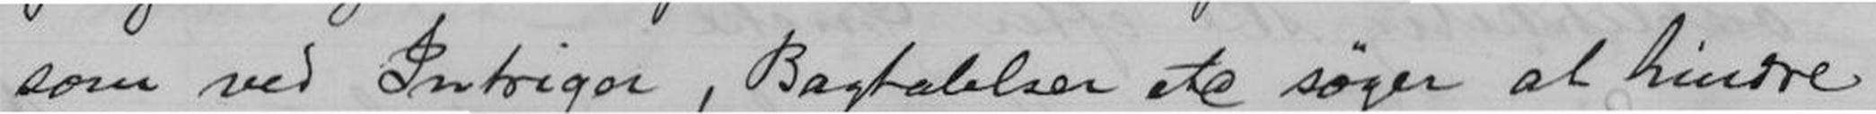som ved Intriger, Bagtaler etc søger at hindre
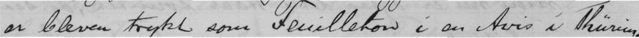er bleven trygt som Feuilleton i en Avis i Thürungen
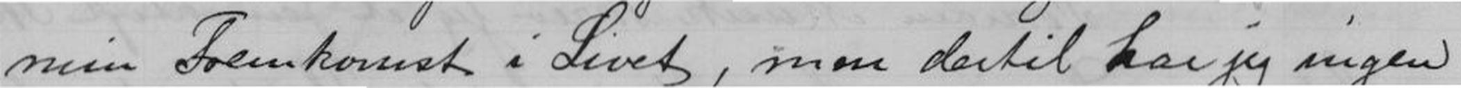min Fremkomst i Livet, men dertil har jeg ingen
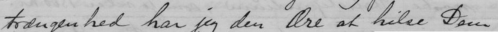trængenhed har jeg den Ære at hilse Dem
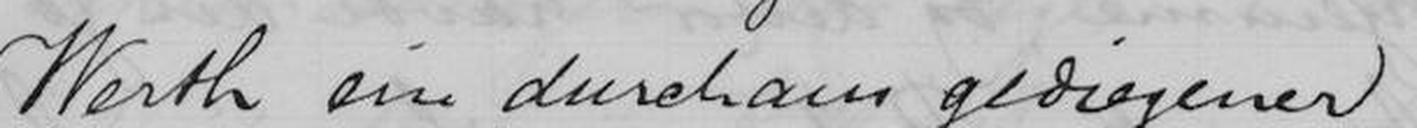Werth ein durcham gediegener."
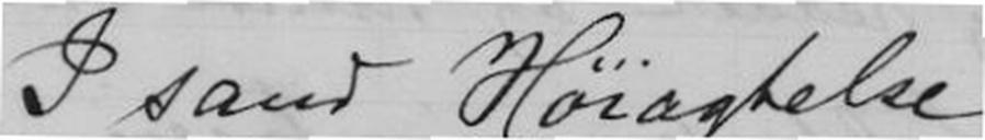I sand Høiagtelse
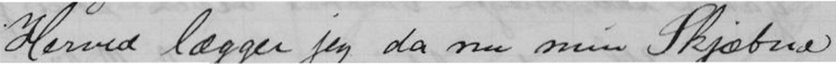Herved lægger jeg da nu min Skjæbne
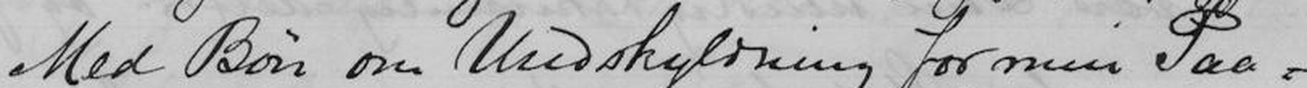Med Bøn om Undskyldning for min Paa-
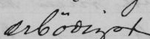ærbødigst
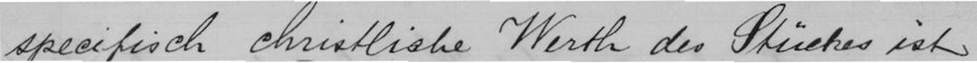specifisch christlishe Werth des Stüches ist
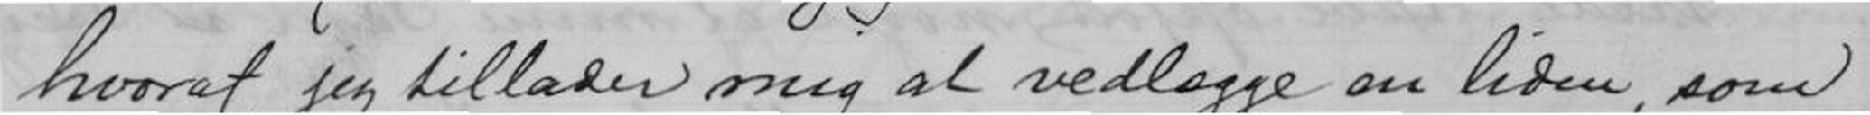hvoraf jeg tillader mig at vedlegge en liden, som
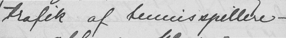trafik af tennisspillere -
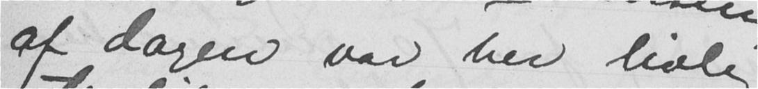af dagen var her livlig
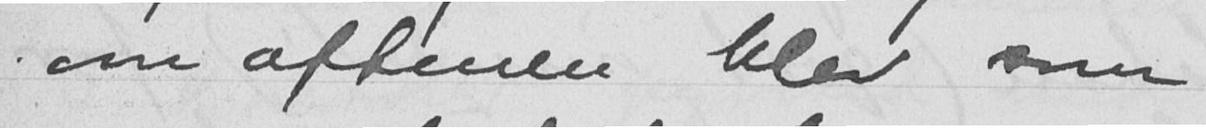om aftenen blev som
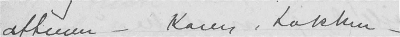aftenen - Karen, Lakken -
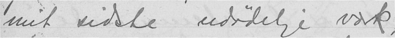mit sidste udødelige værk,
